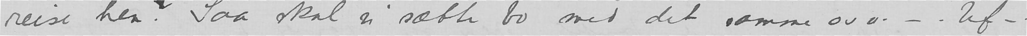reise hen? Saa skal vi sætte bo med det samme osv. –. Uf –.
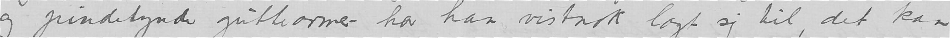og pindetynde guttearmer har han vistnok lagt sig til, det kan
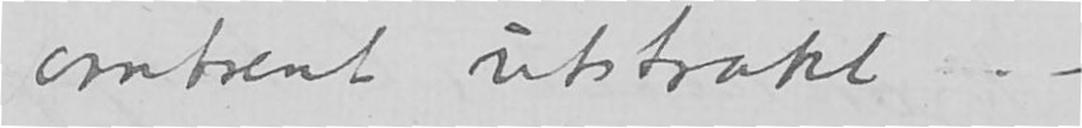omtrent utstrakt – –.
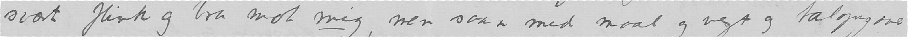svært flink og bra mot mig, men saan med maal og vegt og talopgaver
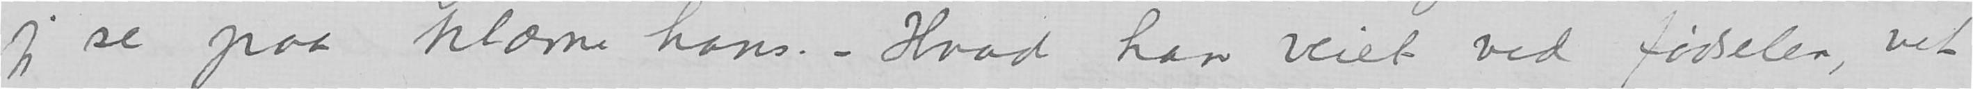jeg se paa klærne hans. – Hvad han veiet ved fødselen, vet
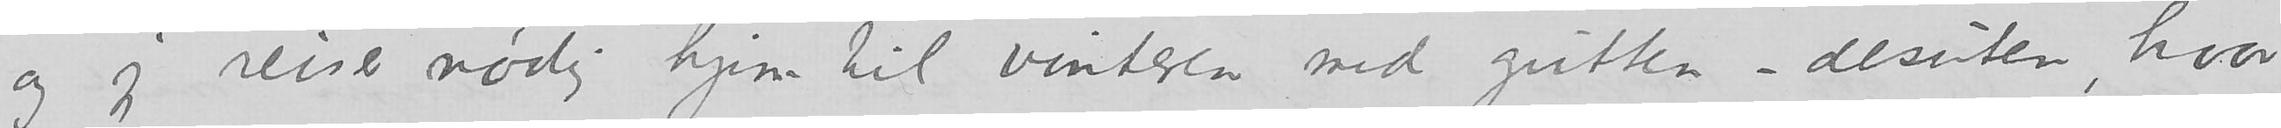og jeg reiser nødig hjem til vinteren med gutten – dessuten, hvor
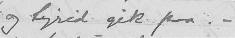og Sigrid gik paa. -
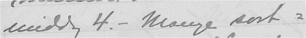middag 4. - Mange sort-
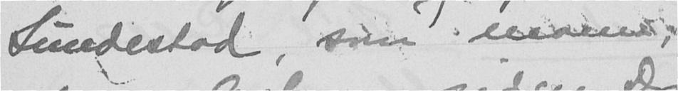Lundestad, som mama,
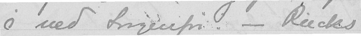i ved Sorgenfri. - Riecks
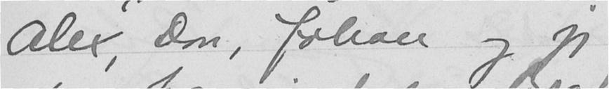Alex, Don, Johan og jeg
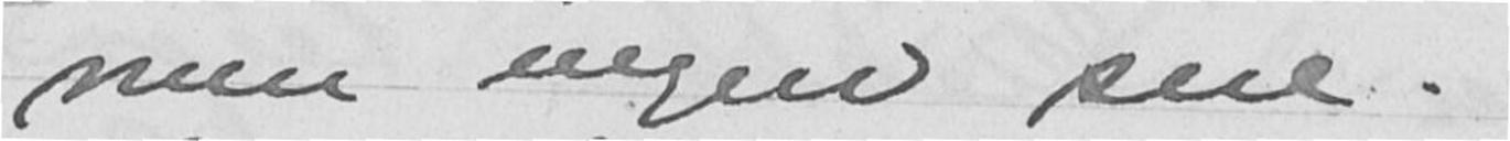men ingen sne.
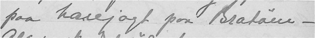paa harejagt paa Bratøen -
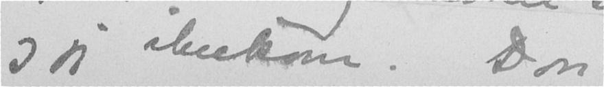og jeg ibnkom. Don
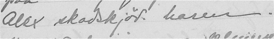Alex skadskjød haren.
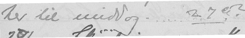her til middag. 27 ?
